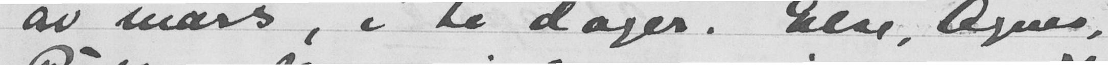av mars, i ti dager. Else, Agnes,
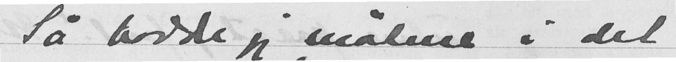Så hadde jeg møtene i det
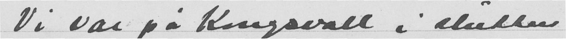Vi var på Kongsvoll i slutten
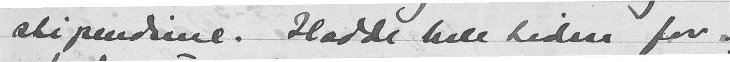stipendiene. Hadde hele tiden for
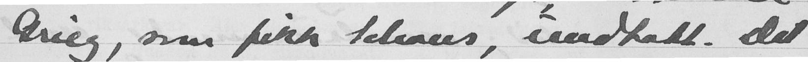Grieg, som fikk Schous, undtatt. Det
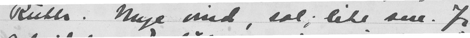Ruth. Mye vind, sol; lite sne. Jeg
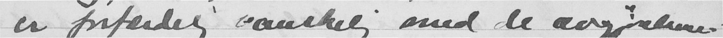er forfærdelig vanskelig med de avgjørelsene
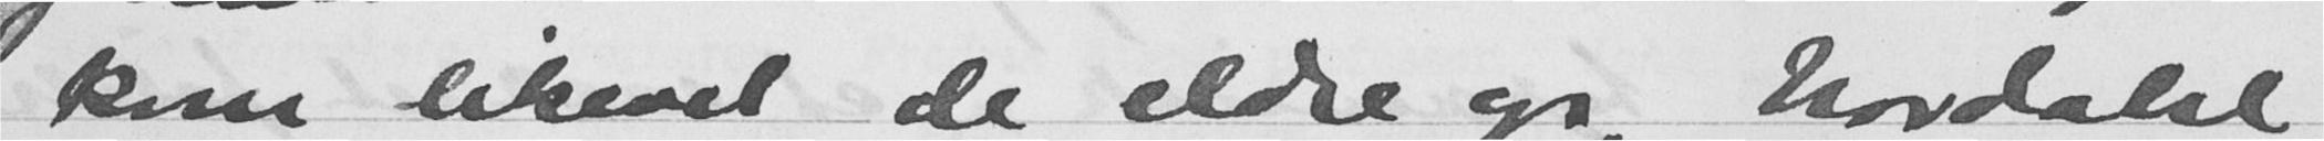kom likevel de eldre også, Nordahl
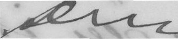Din
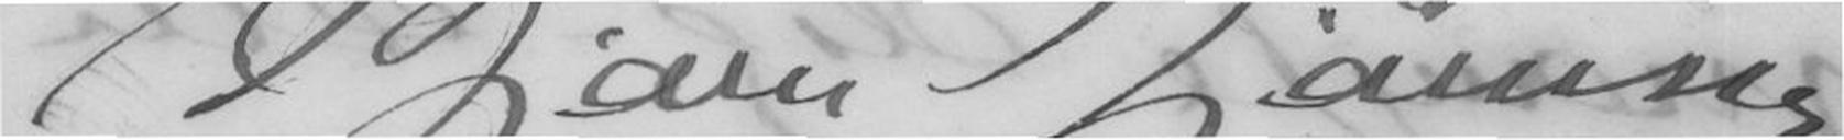Bjørn Bjørnson
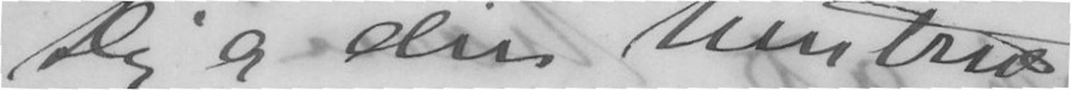Dig og din hustru
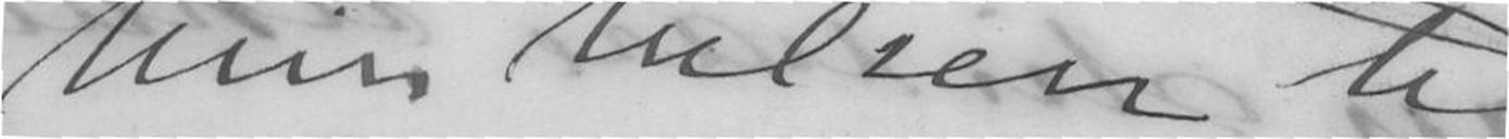Min hilsen til
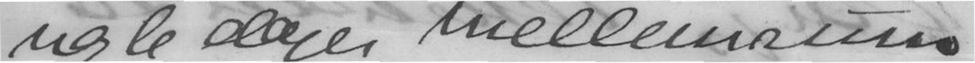nogle dager mellemrum.
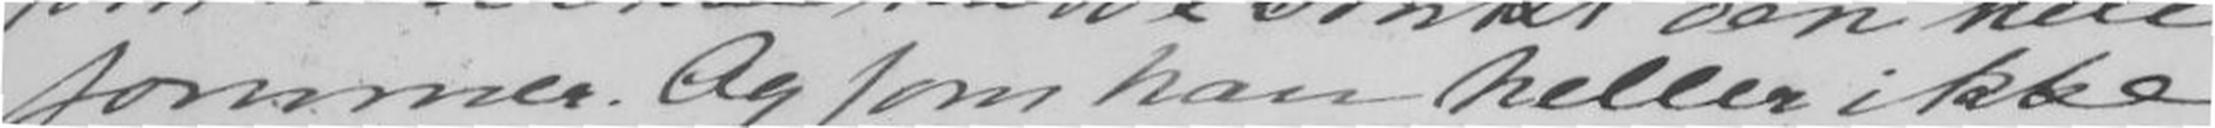sommer. Og som han heller ikke
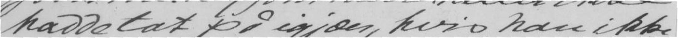hadde tat på igjæn, hvis han ikke
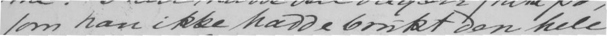som han ikke hadde brukt den hele
